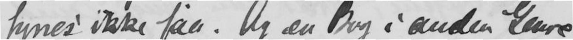synes ikke saa. Og en Bog i anden Genre
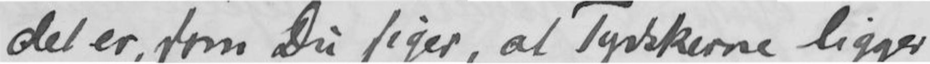det er, som Du siger, at Tydskerne ligger
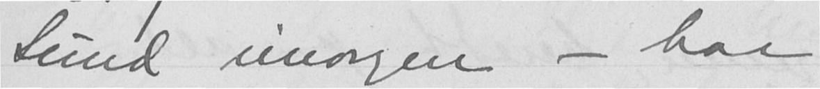Sund imorgen - har
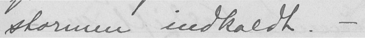stormen indkaldt. -
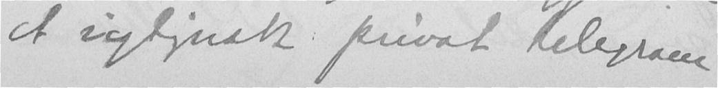et rigtignok privat telegram
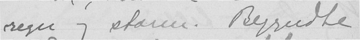regn og storm. Begyndte
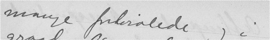mange fortvivlede og i
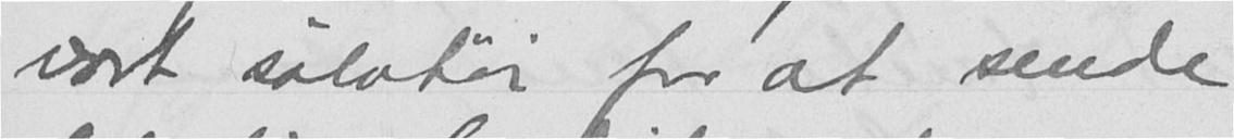vort sølvtøi for at sende
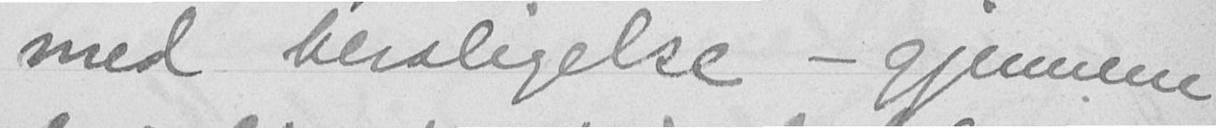med beroligelse - gjennem
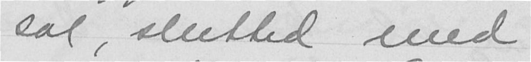sol, slutted med
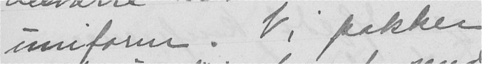uniform. Vi pakker
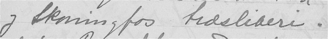og Skoningfos træsliberi.
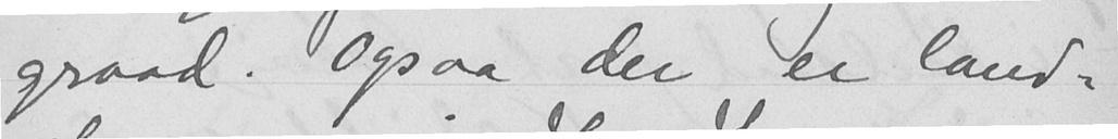graad. Ogsaa der er land-
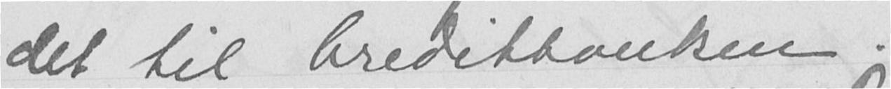det til kreditbanken.
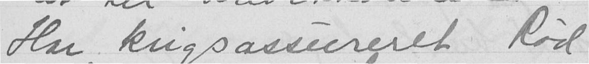Har krigsassureret Rød
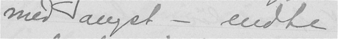med angst - endte
